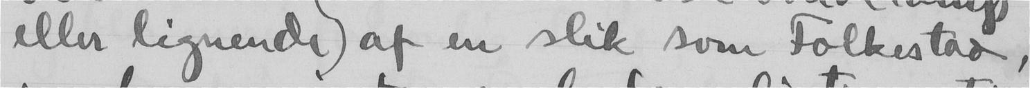eller lignende) af en slik som Folkestad,
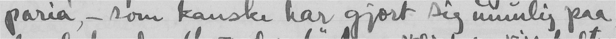paria, - som kanske har gjort sig umulig paa
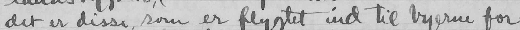det er disse, som er flygtet ind til byerne for
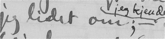jeg lidet om; -
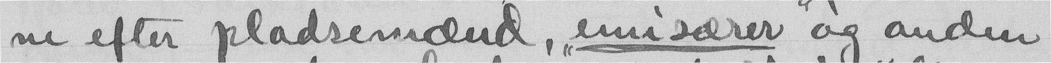ne efter pladsemænd, "emisærer" og anden
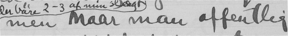men naar man offentlig
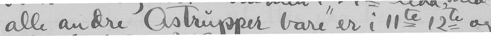alle andre Astrupper bare er i 11te 12te og
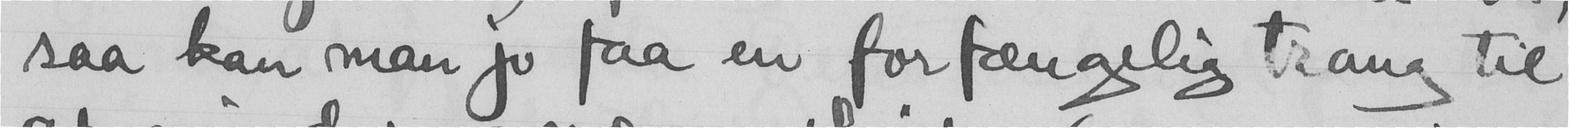saa kan man jo faa en forfængelig trang til
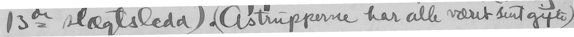13de slægtsledd). (Astrupperne har alle været sent gifte)
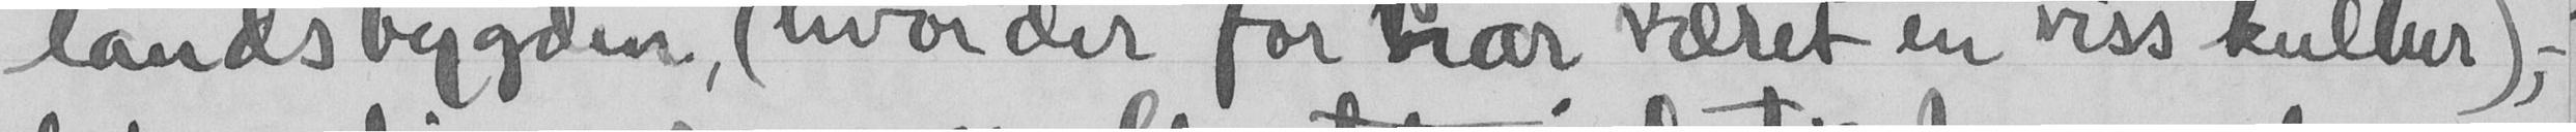landsbygden, (hvor der før har været en viss kultur),-
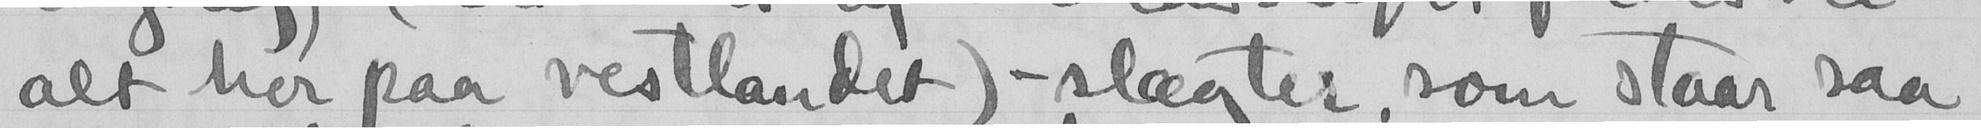alt her paa vestlandet) - slægter, som staar saa
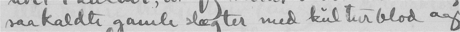saakaldte gamle slægter med kulturblod og
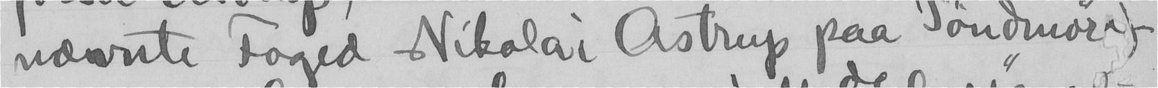nævnte Foged Nikolai Astrup paa Søndmøre)
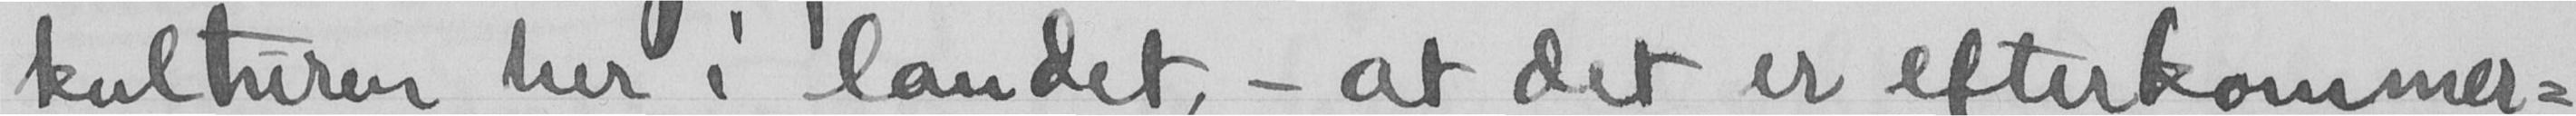kulturen her i landet, - at det er "efterkommer-
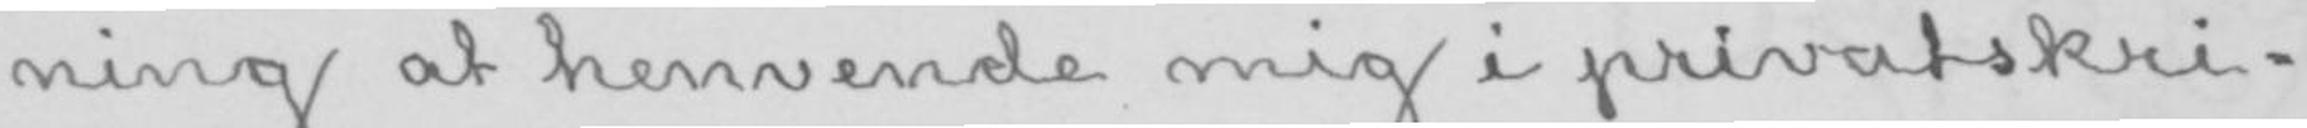ning at henvende mig i privatskri-
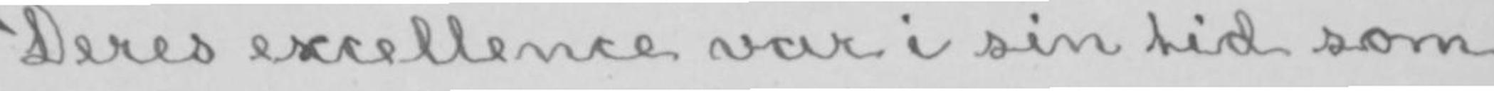Deres excellence var i sin tid som
